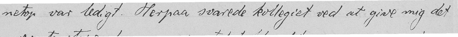netop var ledigt. Herpaa svarede kollegiet ved at give mig det
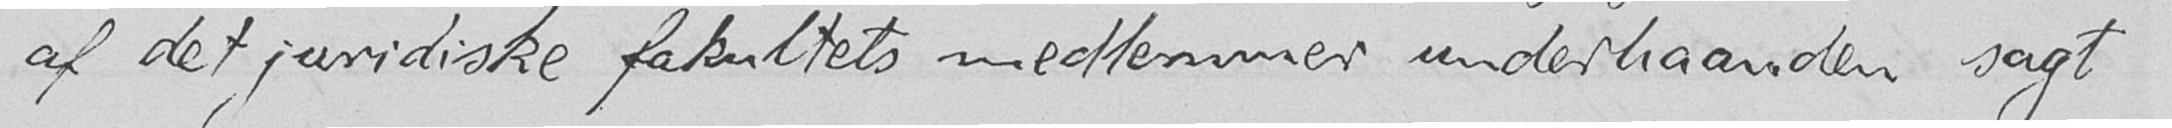af det juridiske fakultets medlemmer underhaanden sagt
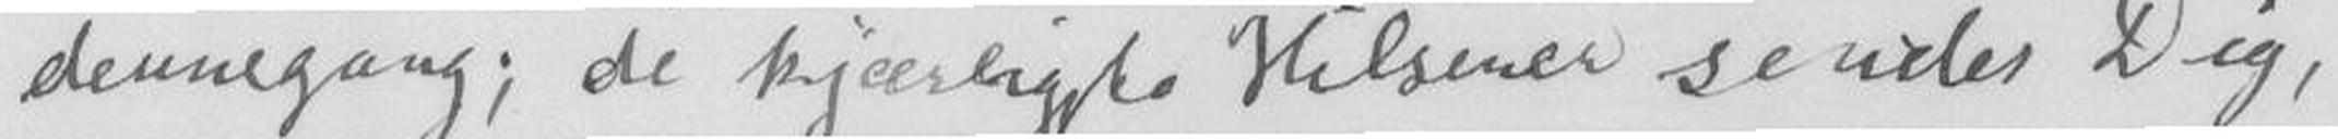dennegang; de kjærligste Hilsener sendes Dig,
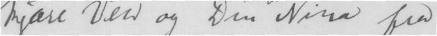kjære Ven og Din Nina fra
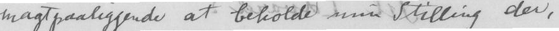magtpaaliggende at beholde min Stilling der,
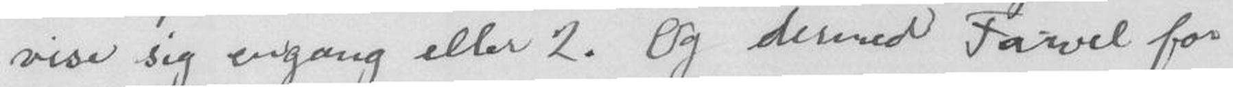vise sig engang eller 2. Og dermed Farvel for
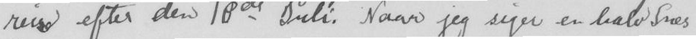reise efter den 18de Juli. Naar jeg siger en halv Snes
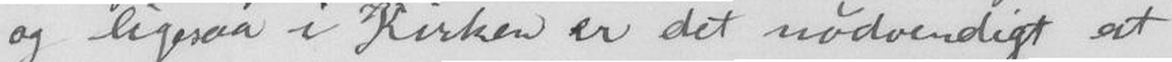og ligesaa i Kirken er det nødvendigt at
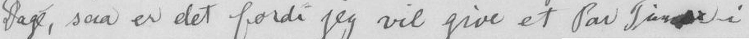Dage, saa er det fordi jeg vil give et Par Timer i
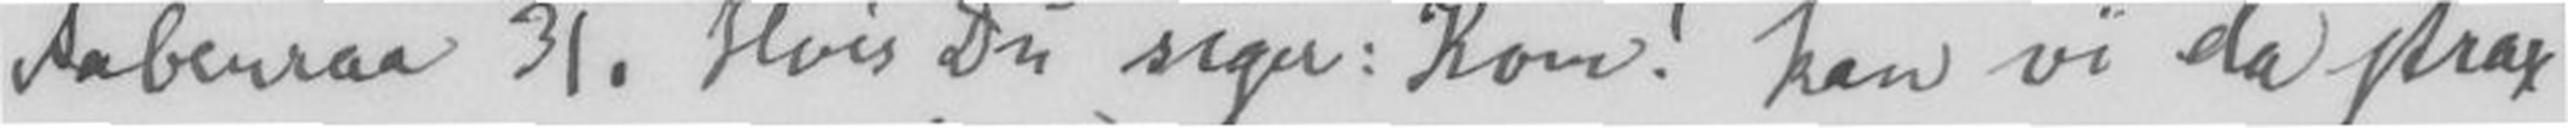Aabenraa 31. Hvis Du siger: Kom! kan vi da strax
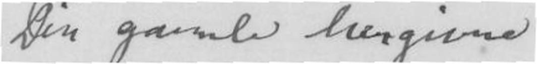Din gamle hengivne
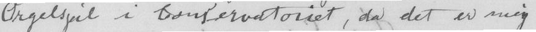Orgelspil i Conservatoriet, da det er mig
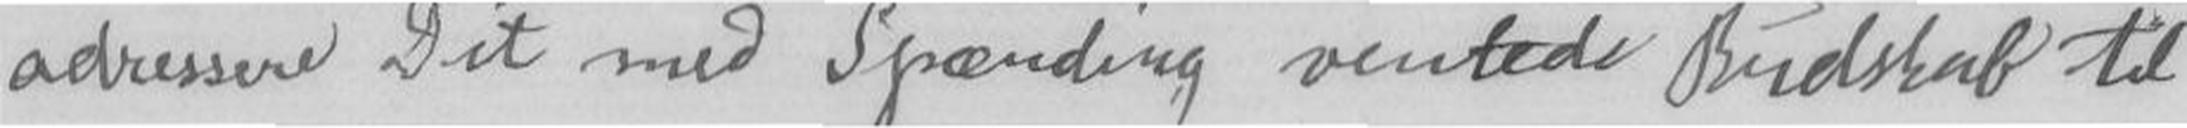adressere Dit med Spænding ventede Budskab til
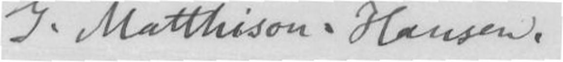G. Matthison=Hansen.
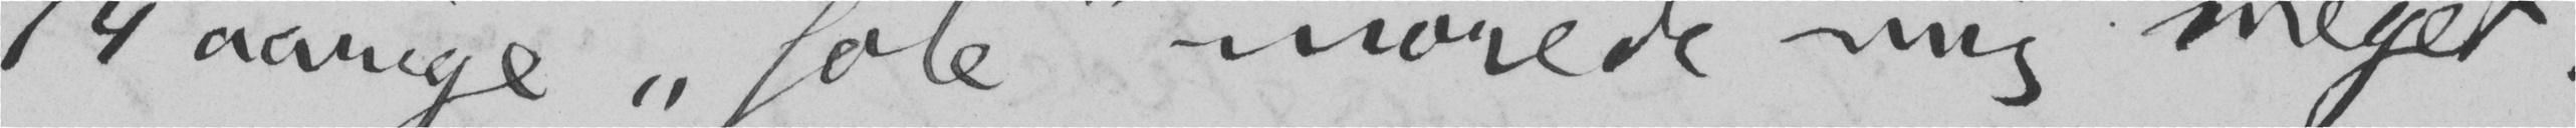74 Aarige «fole» morede mig meget.
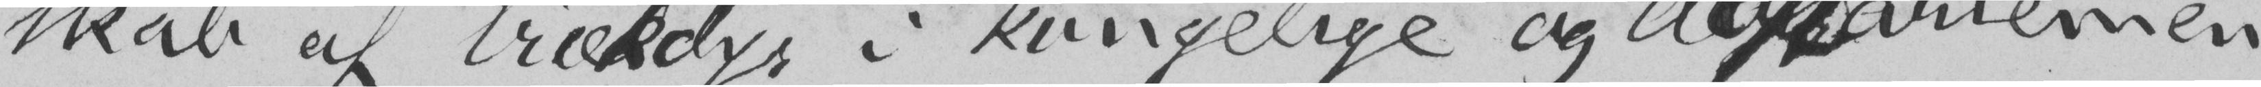skab af trækdyr i kongelige og departemen-
